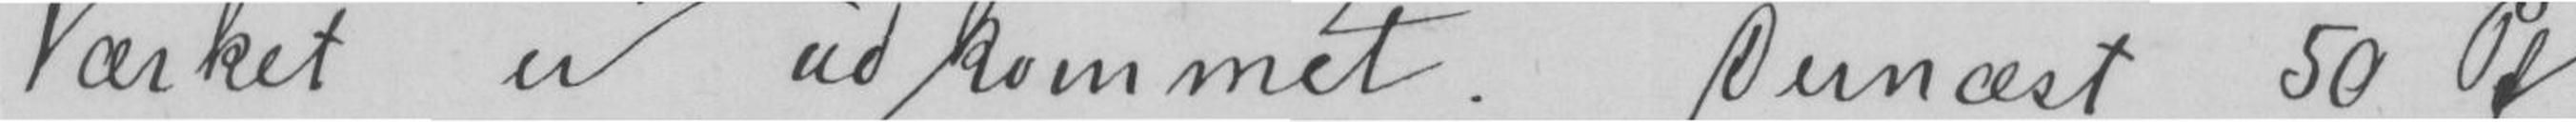Værket er udkommet. Dernæst 50 Pf
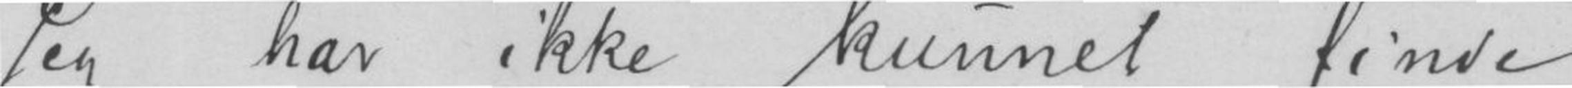Jeg har ikke kunnet finde
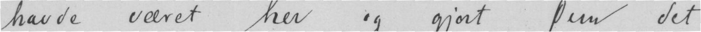havde været her og gjort dem det
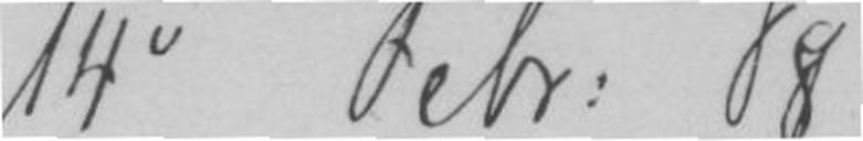14 Febr. 88
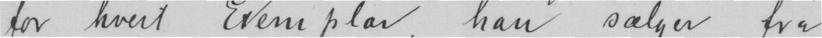for hvert Exemplar, han sælger fra
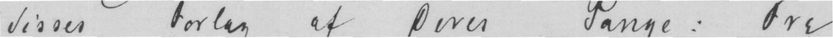disser Forlag af Deres Sange: Fra
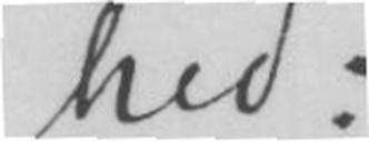hed:
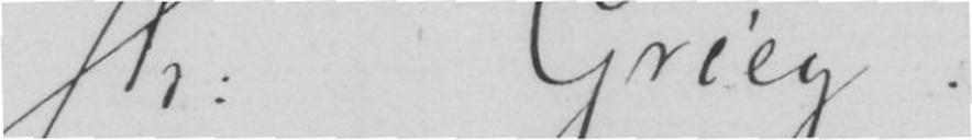Hr. Grieg!
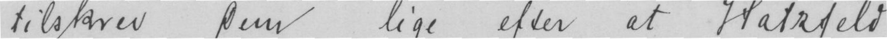tilskrev dem lige efter at Hatzfeld
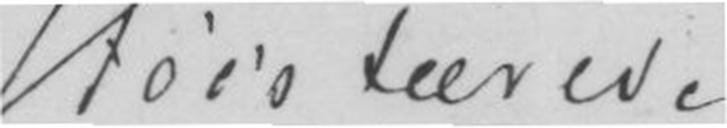Høistærede
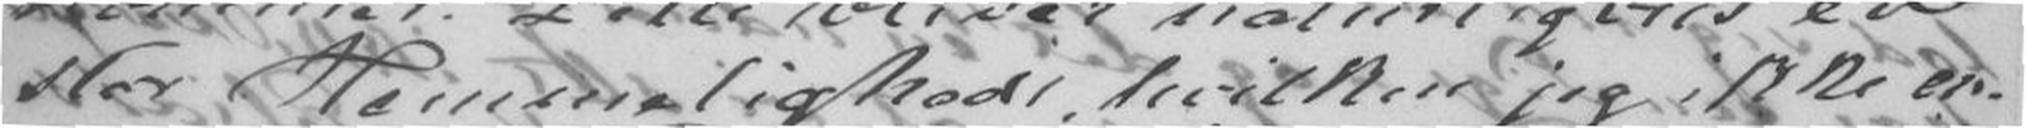stor Hemmelighed, hvilken jeg ikke en-
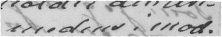medens i mod-
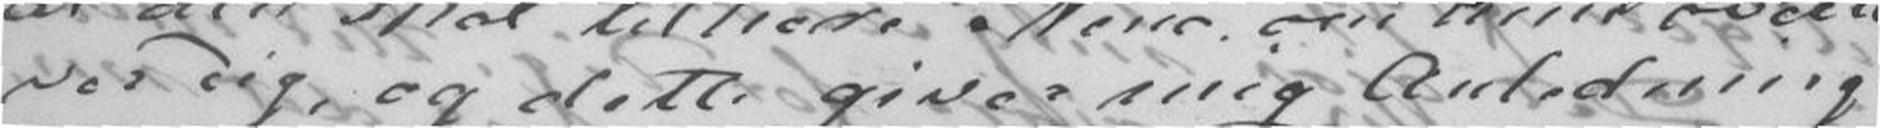ver Dig, og dette giver mig Anledning
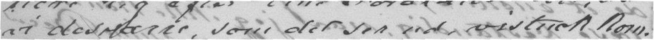vi desværre, som det ser ud, vistnok kom-
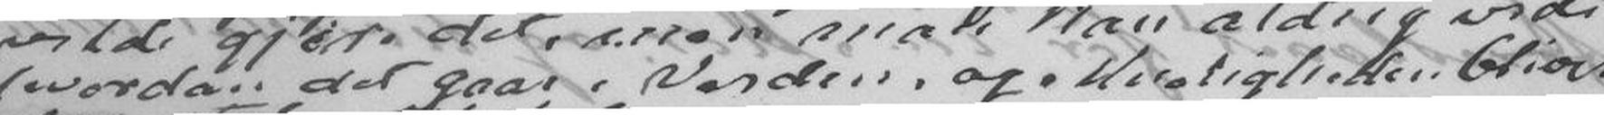hvordan det gaar i Verden, og Mueligheden bliver
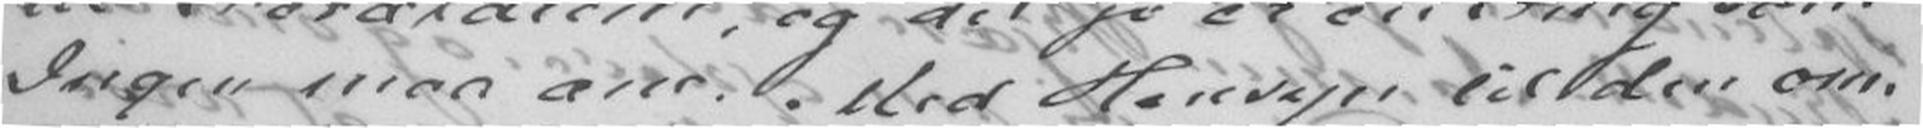Ingen maa ane. Med Hensyn til den om-
